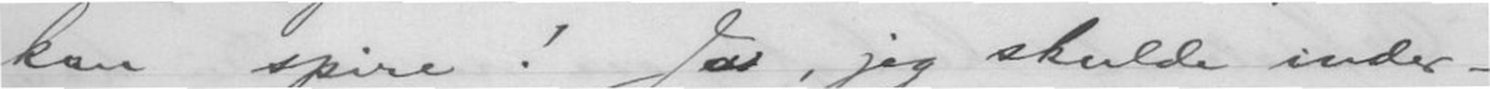kan spire! Ja, jeg skulde inder-
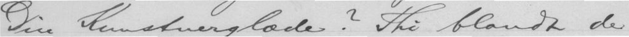Din Kunstnerglæde? Thi blandt de
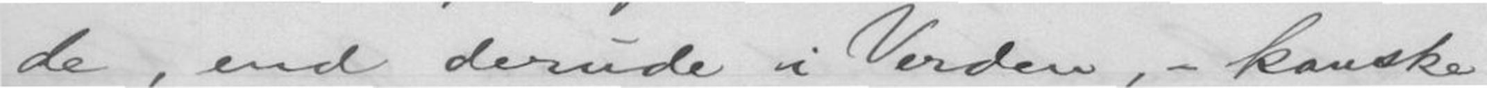de, end derude i Verden, - kanske
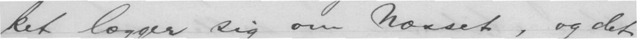ket lægger sig om Næsset, og det
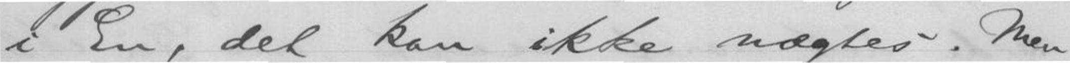i En, det kan ikke nægtes. Men
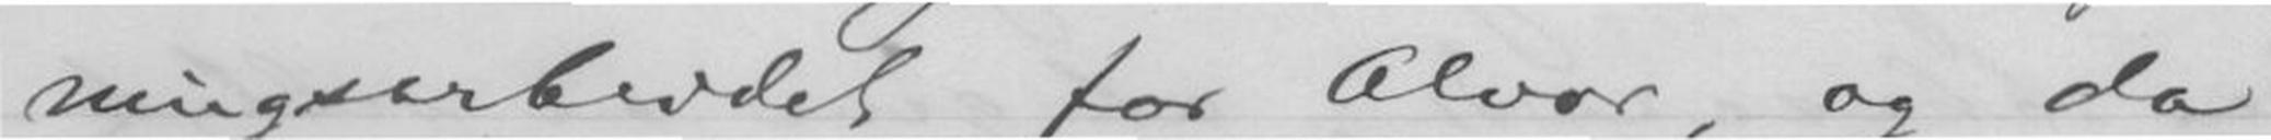ningsarbeidet for Alvor, og da
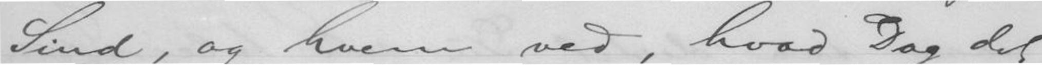Sind, og hvem ved, hvad Dag det
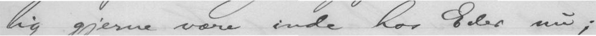lig gjerne være inde hos Eder nu;
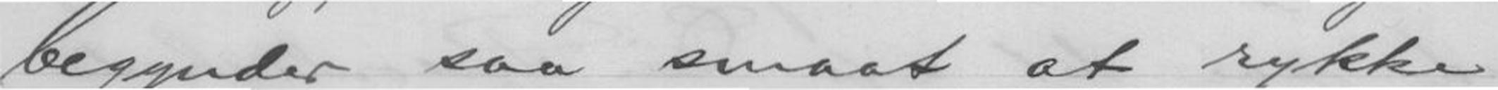begynder saa smaat at rykke
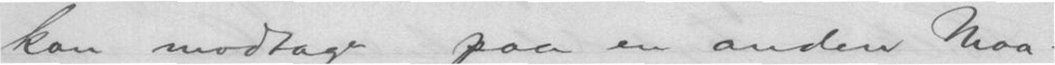kan modtage paa en anden Maa-
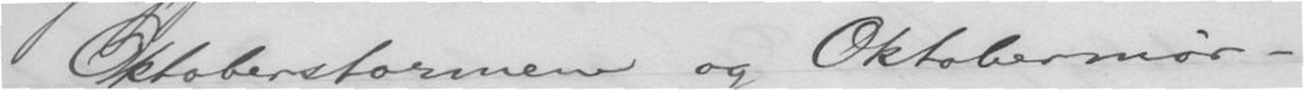Oktoberstormene og Oktobermør-
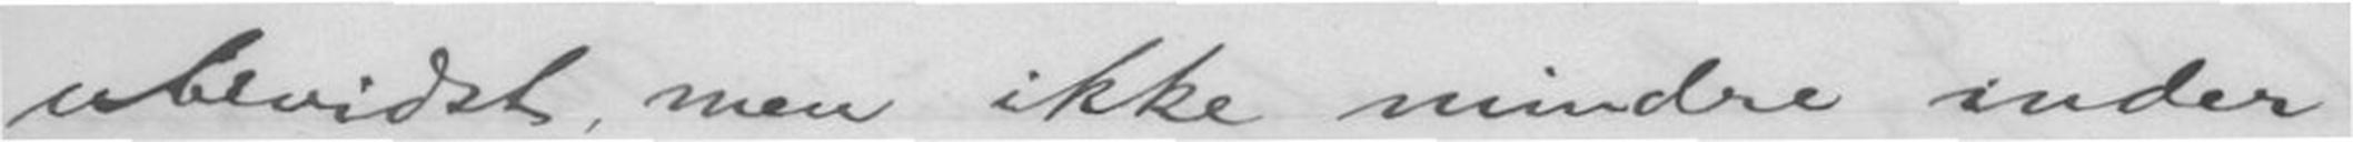ubevidst, men ikke mindre inder-
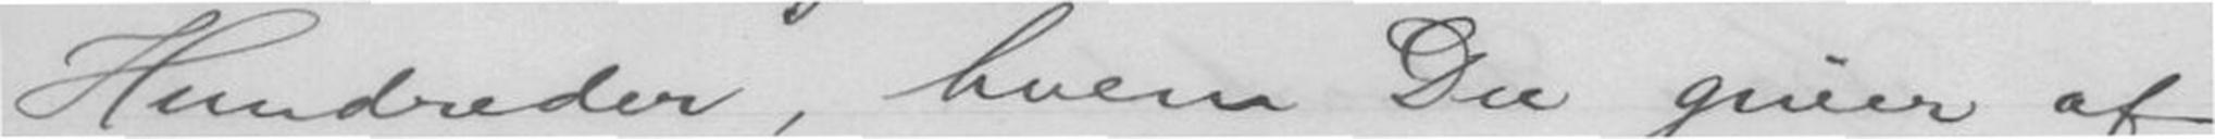Hundreder, hvem Du giver af
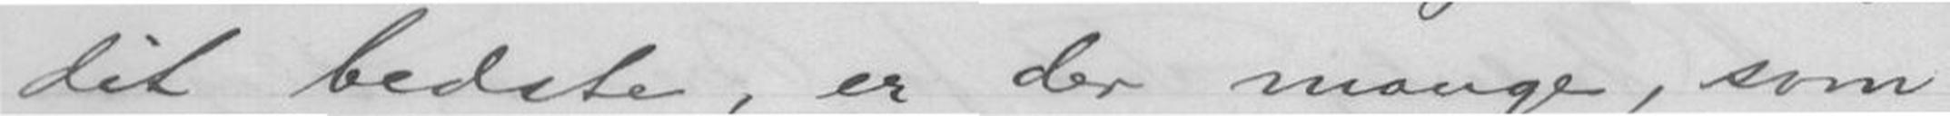dit bedste, er der mange, som
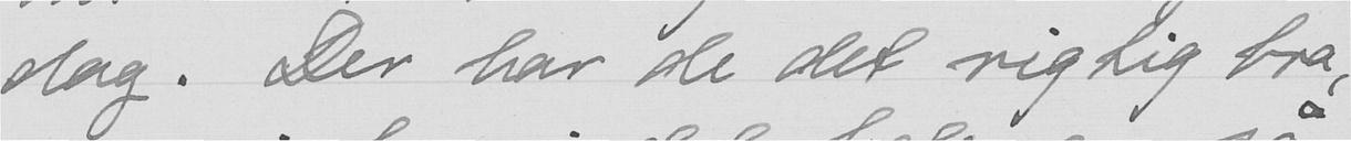dag. Der har de det rigtig bra,
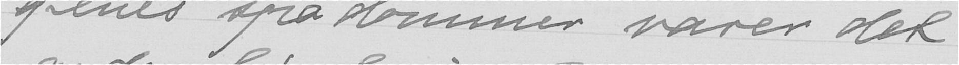genes spådommer varer det
